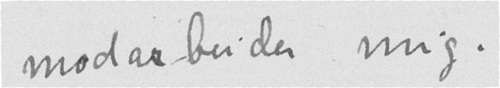modarbeider mig.
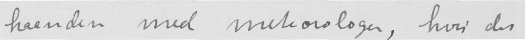haanden med meteorologen, hvis der
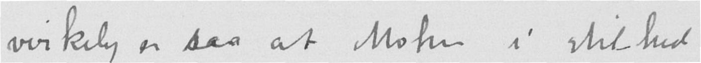virkelig er saa at Motre i stilhed
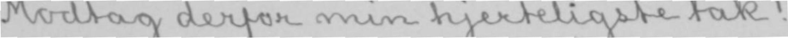Modtag derfor min hjerteligste tak!
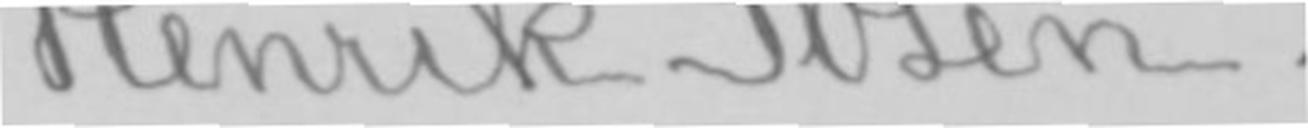Henrik Ibsen.
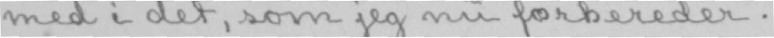med i det, som jeg nu forbereder.
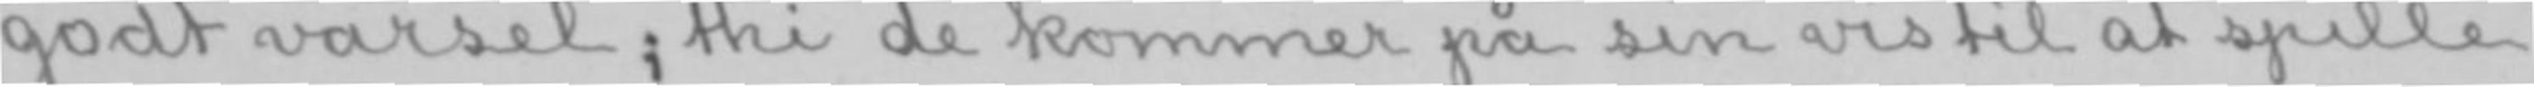godt varsel; thi de kommer på sin vis til at spille
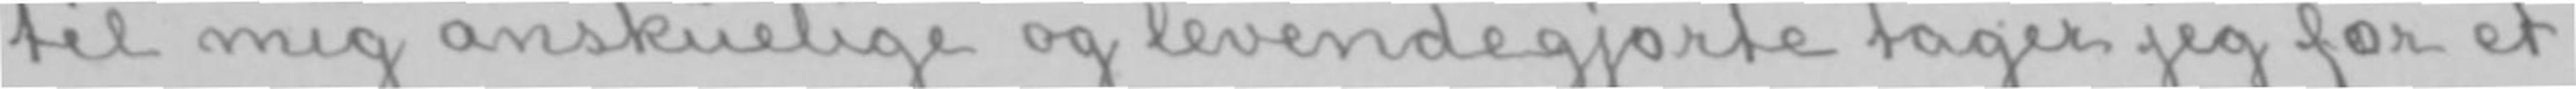til mig anskuelige og levendegjorte tager jeg for et
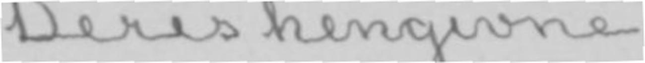Deres hengivne
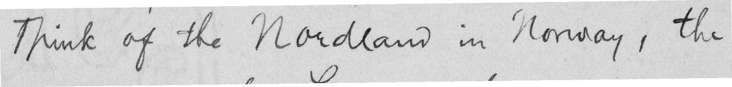Think of the Nordland in Norway, the
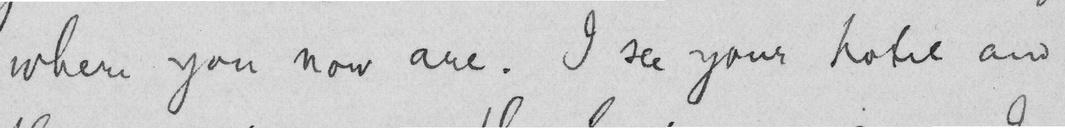where you now are. I see your hotel and
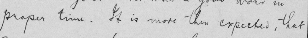proper time. It is more than expected, that
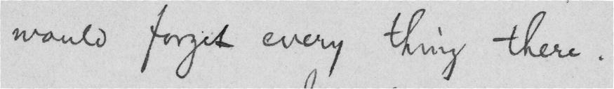would forget every thing there.
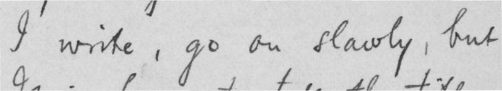I write, go on slowly, but
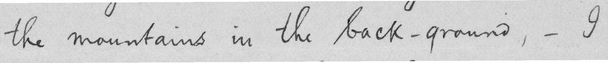the mountains in the back-ground, - I
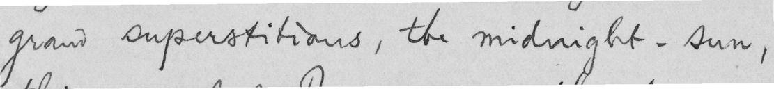grand superstitions, the midnight-sun,
